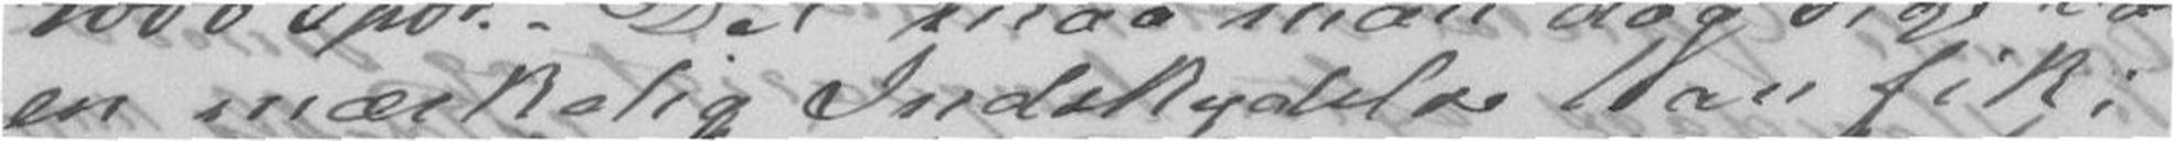en mærkelig Indskydelse han fik;
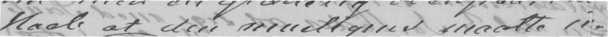Haab at den mueligens maatte in-
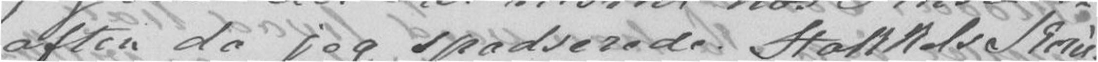aften da jeg spadserede. Stakkels Kone,
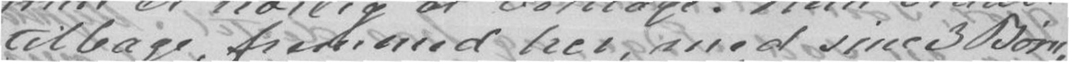tilbage, fremmed her, med sime 3 Børn,
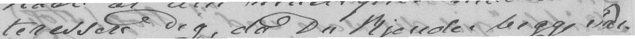teressere Dig, da Du kjende begge Par-
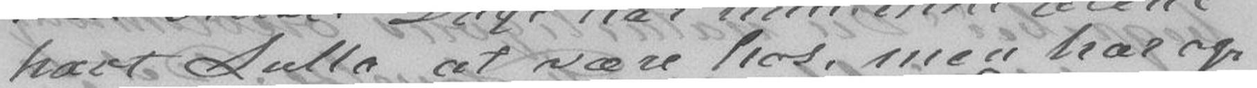havt Lulla at være hos, men har og-
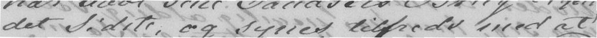det Sidste, og synes tilfreds med at
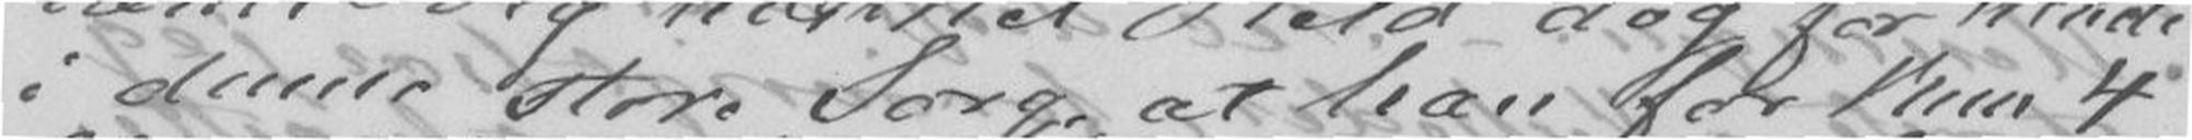i denne store Sorg at han for kun 4
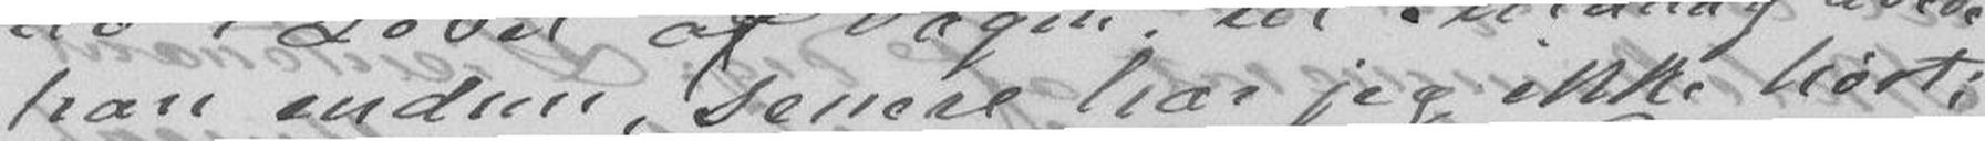han endnu, senere har jeg ikke hørt;
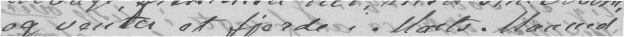og venter et fjerde i Marts Maaned
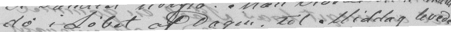dø i Løbet af Dagen, til Middag levede
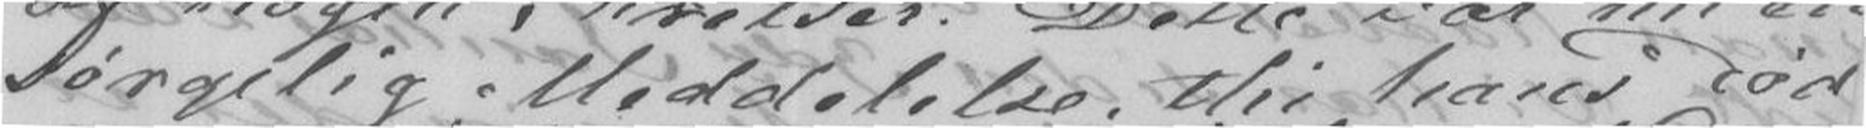sørgelig Meddelse, thi hans Død
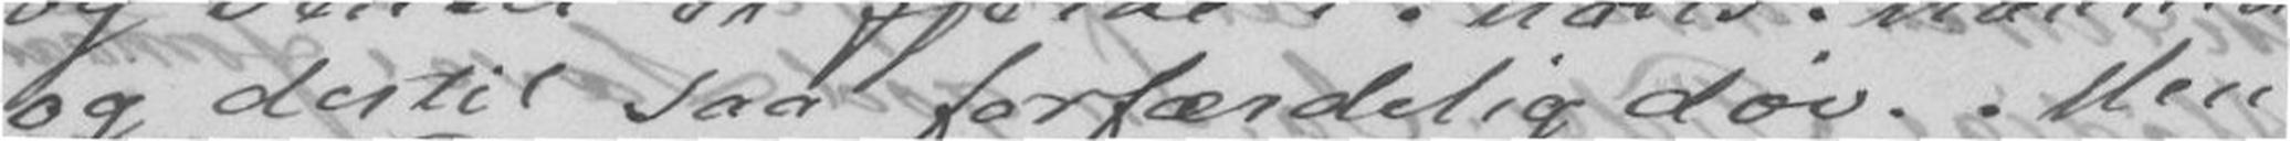og dertil saa forfærdelig døv. Men
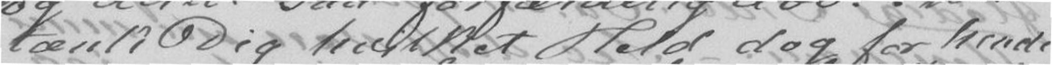tænk Dig hvilket Held dog for hende
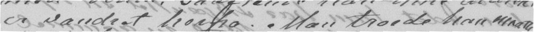er vandret herfra. Man troede han maatte
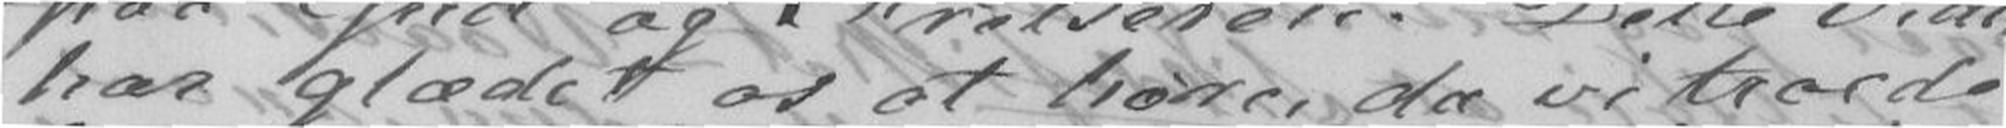har glædet os at høre, da vi troede
